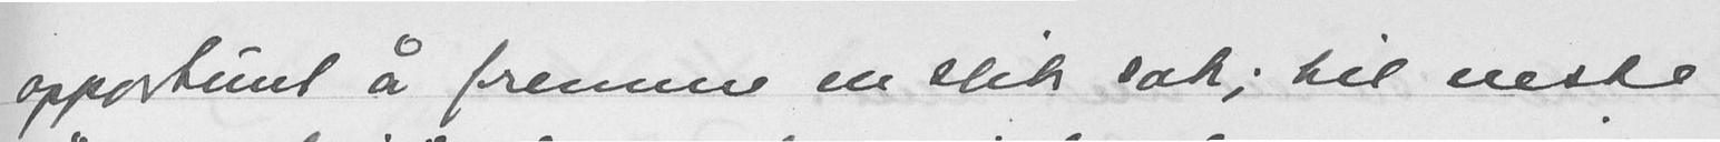opportunt å fremme en slik sak; til neste
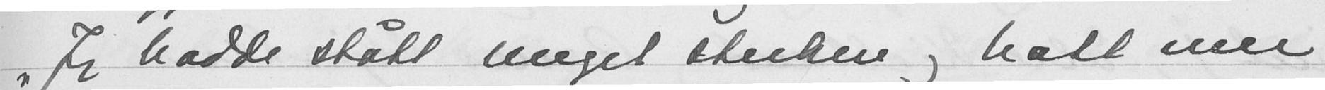"Jeg hadde stått meget sterkere og hatt mer
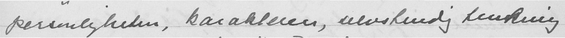personligheten, karakteren, selvstendig tenkning
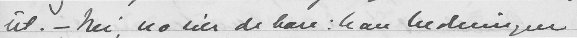ut. - Nei, no sier de bare: han hedningen
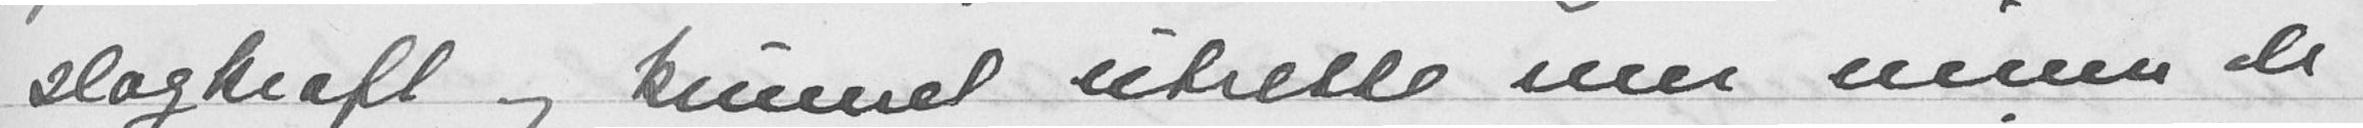slagkraft og kunnet utrette mer innen de
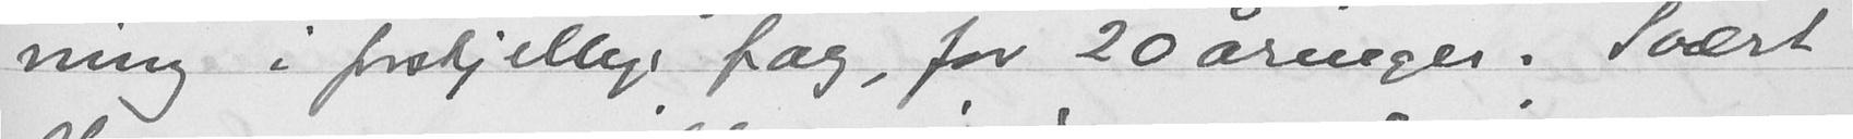ning i forstjellig fag for 20 åringer. Svært
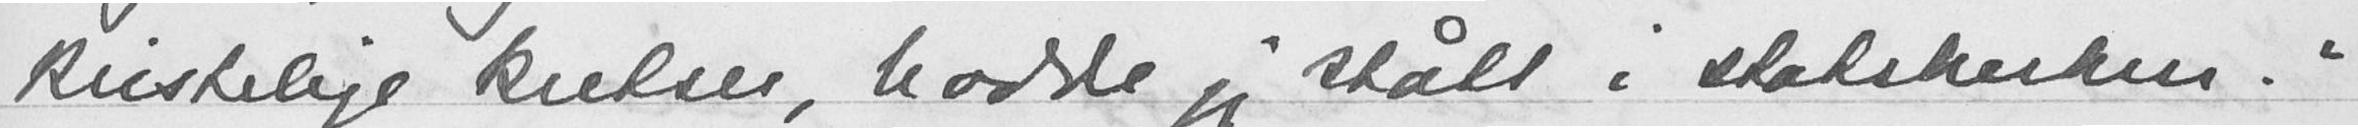kristelige kretser, hadde jeg stått i statskirken."
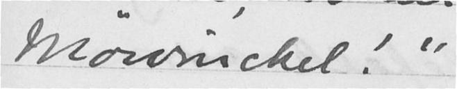Mowinckel!!
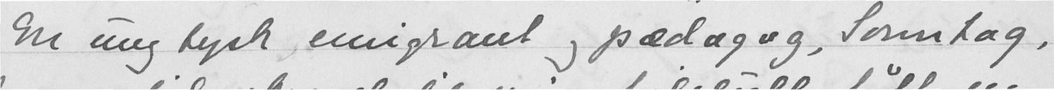En ung tysk emigrant og pædagag "Sorentag",
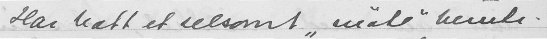Har hatt et selsomt "møte" herute.
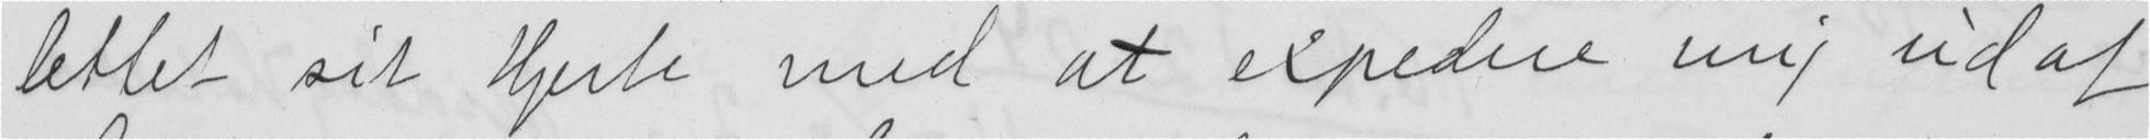lettet sit Hjerte med at expedere mig ud af
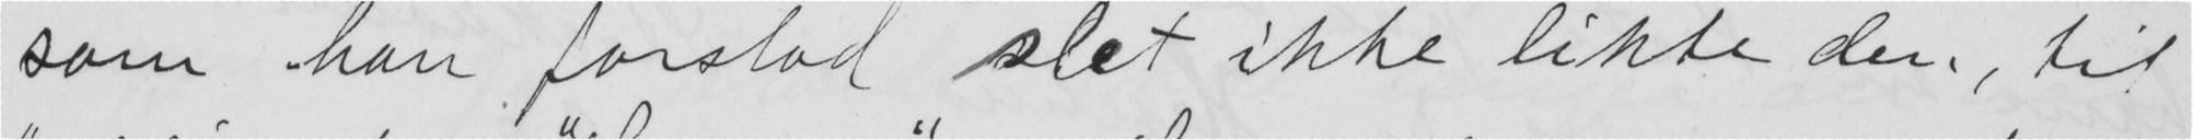som han forstod slet ikke likte den, til
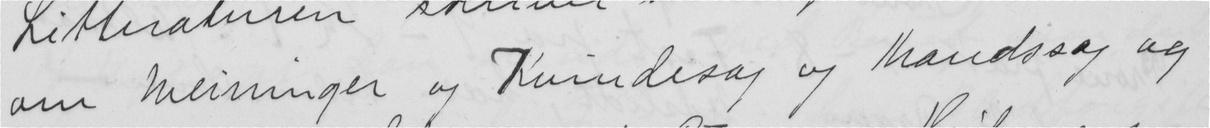om Meininger og Kvindesag og Mandssag og
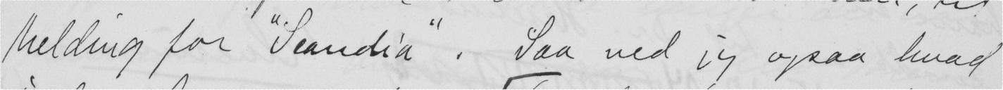Melding for "Scandia". Saa ved jeg ogsaa hvad
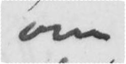om
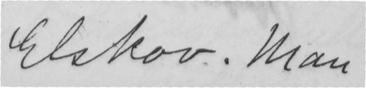Elskov. Man
